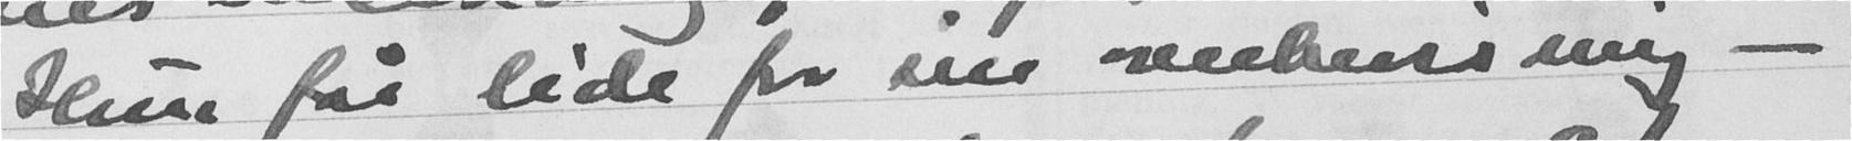Hun får lide for sin overbevisning -
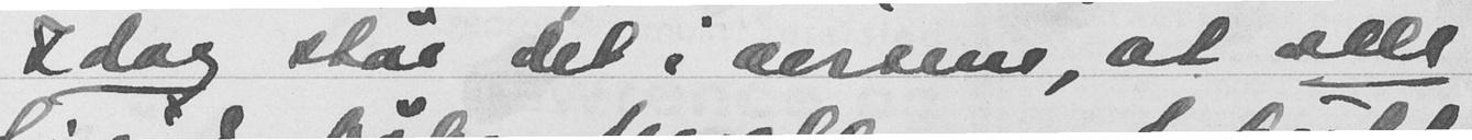I dag står det i avisene, at alle
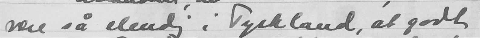være så elendig i Tyskland, at godt
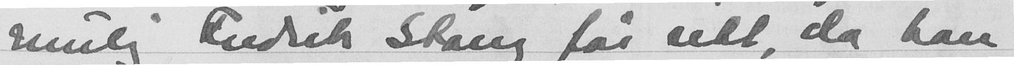mulig Fredrik Stang får rett, da han
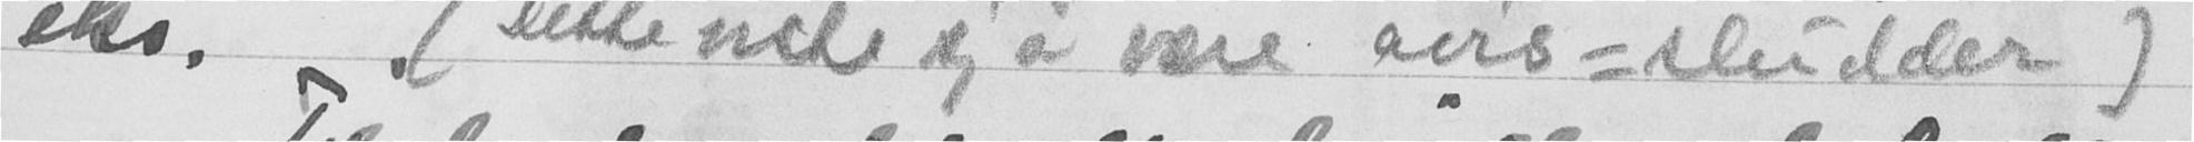eks. (Dette viste sig å være avis-sludder)
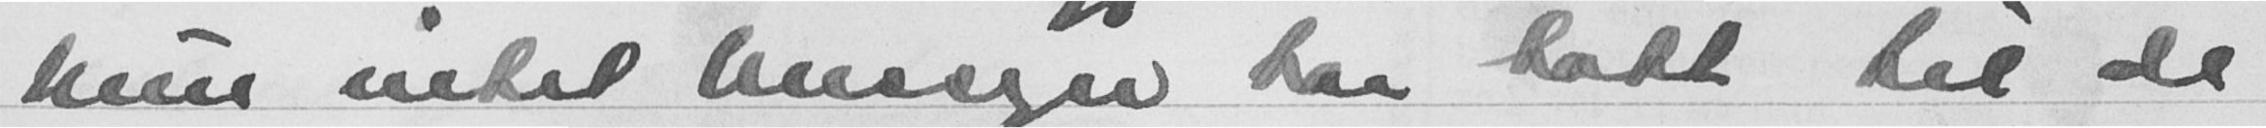hun intet hensyn har tatt til de
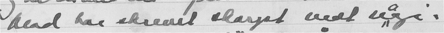blad har skrevet skarpt mot møge-
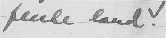fleste land.
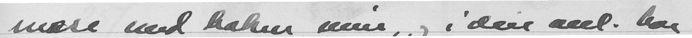mase med boken min, og i den anl. har
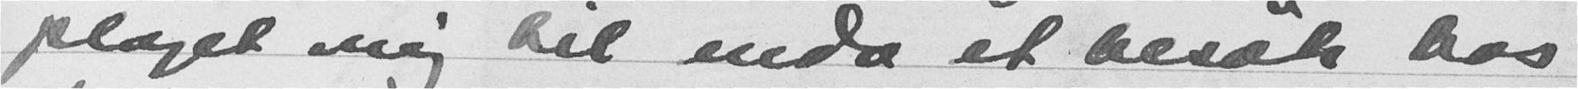plaget mig til enda et besøk hos
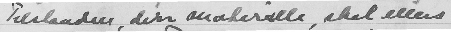Tilstanden, den materielle, skal ellers
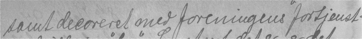samt decoreret med foreningens "fortjenst-
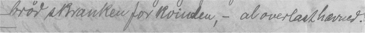- brød skranken for kvinden, - al overlast hævned.
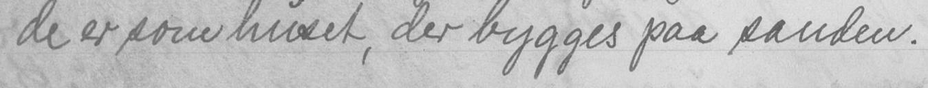de er som huset, der bygges paa sanden.
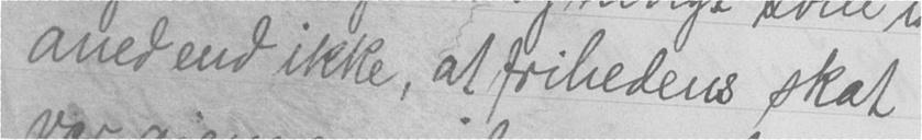aned end ikke, at frihedens skat
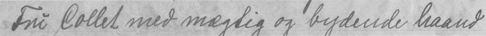Fru Collet med mægtig og bydende haand
